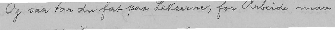Og saa tar du fat paa Lekserne, for Arbeide maa
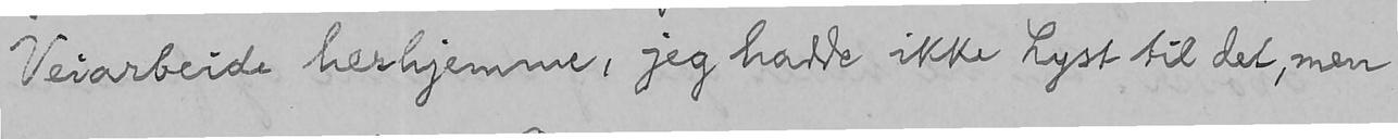Veiarbeide herhjemme, jeg hadde ikke Lyst til det, men
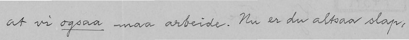at vi ogsaa maa arbeide. Nu er du altsaa slap,
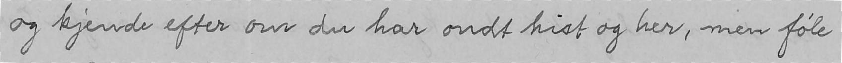og kjende efter om du har ondt hist og her, men føle
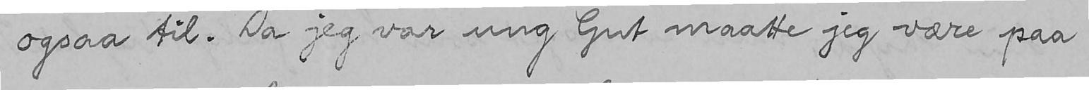ogsaa til. Da jeg var ung Gut maatte jeg være paa
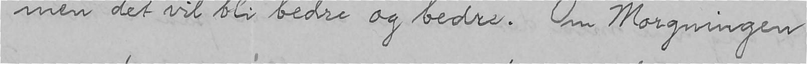men det vil bli bedre og bedre. Om Morgningen
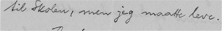til Skolen, men jeg maatte leve.
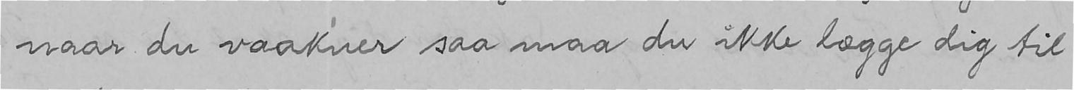naar du vaakner saa maa du ikke lægge dig til
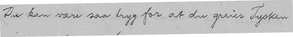Du kan være saa tryg for at du greier Tysken
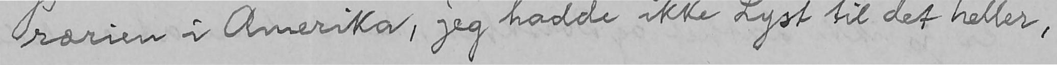Prærien i Amerika, jeg hadde ikke Lyst til det heller,
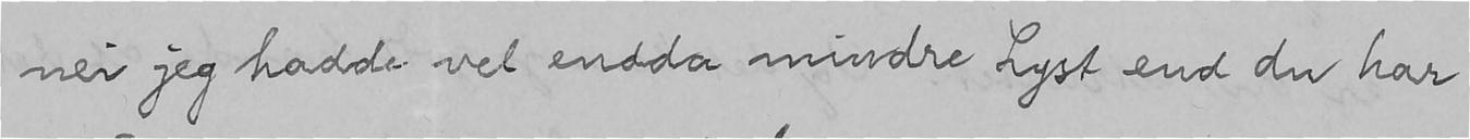nei jeg hadde vel endda mindre Lyst end du har
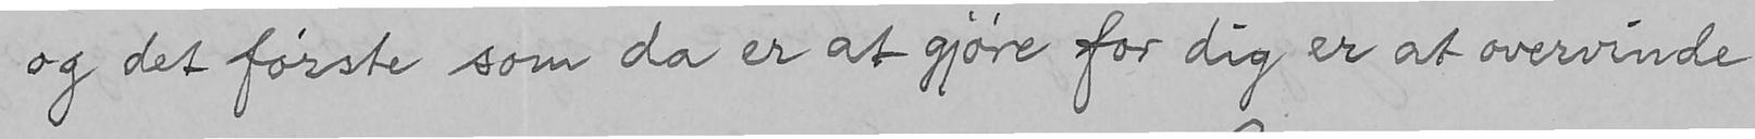og det første som da er at gjøre for dig er at overvinde
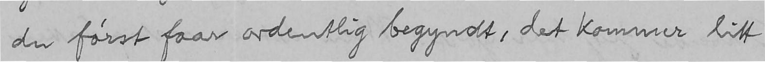du først faar ordentlig begyndt, det kommer litt
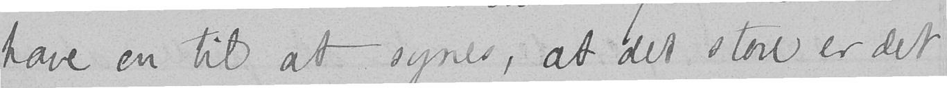have en til at synes, at det store er det
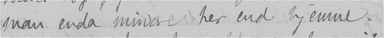man enda mindre her end hjemme.
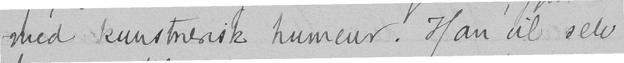med kunstnerisk humeur. Han vil selv
